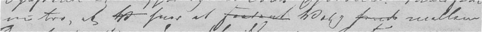nu tro, at V hvor et saadant Valg finde mellem
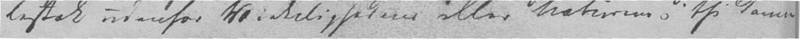lestok udenfor Virkelighedens eller Naturens; thi denne
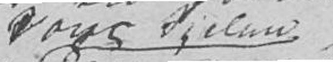som Sjelen
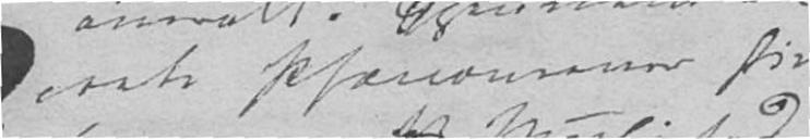crete Phænomener fin-
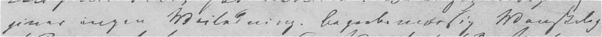giver ingen Veiledning. Begrebsmæssig Vanskelig-
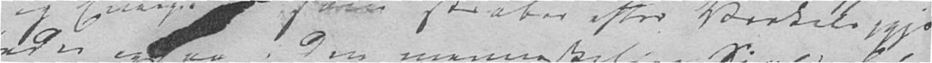og Energie som stræber efter Virkeliggjø-
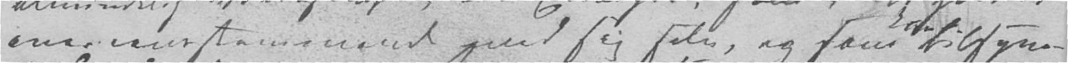overeensstemmende med sig selv, og som tilsyne-
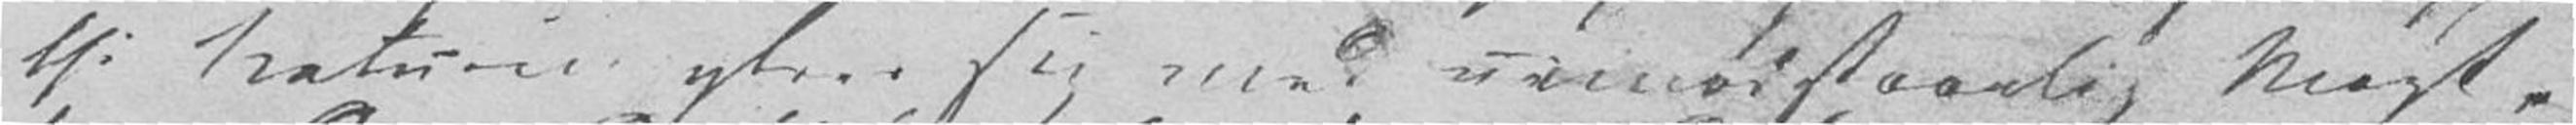thi Naturen ytrer sig med uimodstaaelig Magt.
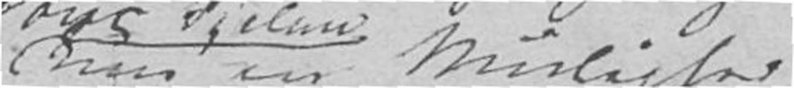kun en Mulighed,
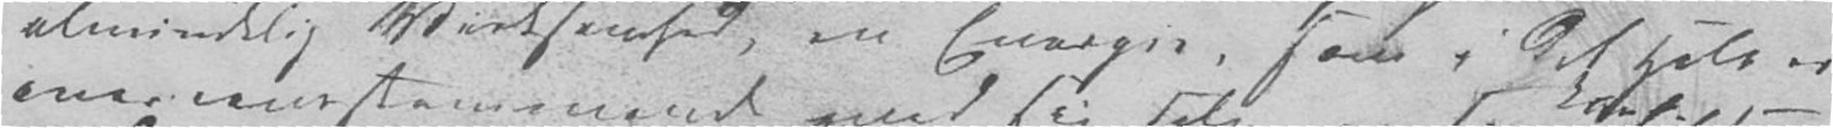almindelig Virksomhed, en Energie, som i det Hele er
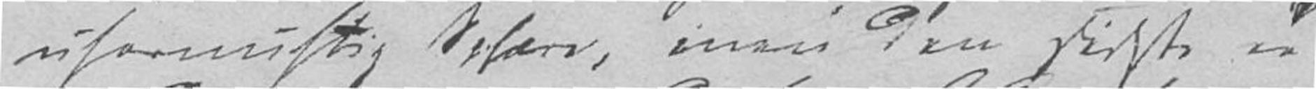ufornuftig Sphære, men den sidste er
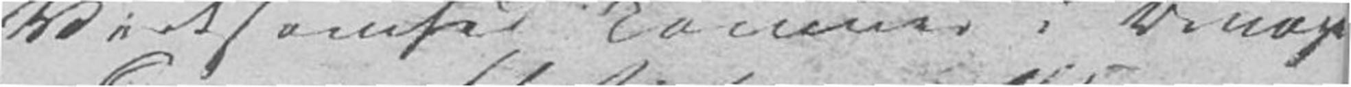Virksomhed kommer i Bevægel-
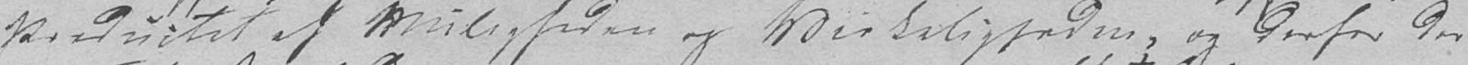Productet af Muligheden og Virkeligheden, og derfor deres
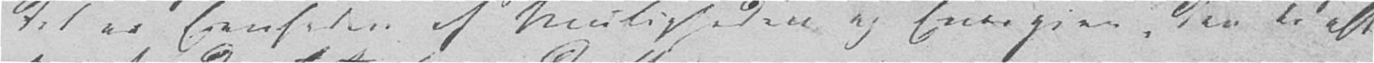det er Eenheden af Muligheden og Energien. Den er alt-
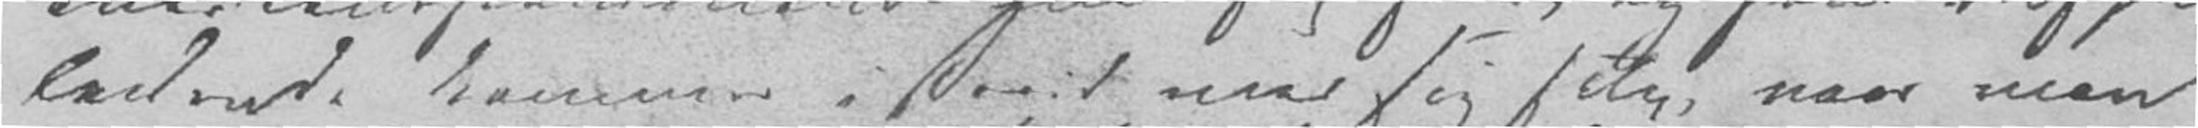ladende kommer i Strid med sig selv, naar man
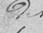Det
